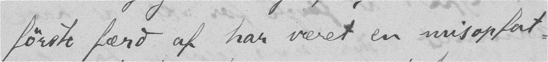første færd af har været en misopfat-
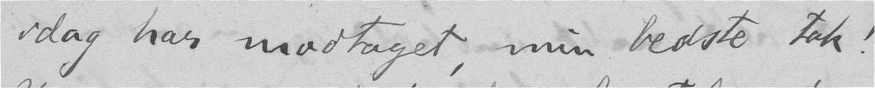idag har modtaget, min bedste tak!
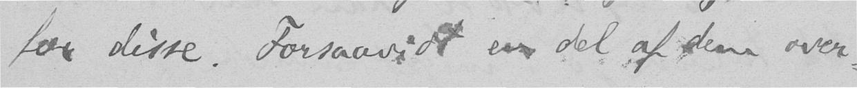for disse. Forsaavidt en del af dem over-
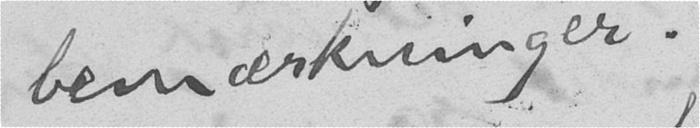bemærkninger.
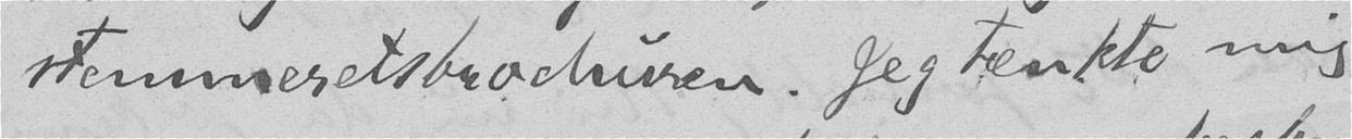stemmeretsbrochuren. Jeg tænkte mig
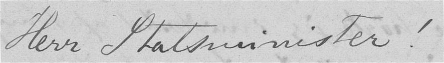Herr Statsminister!
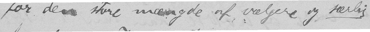for den store mængde af vælgere og særlig
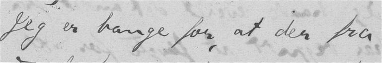Jeg er bange for, at der fra
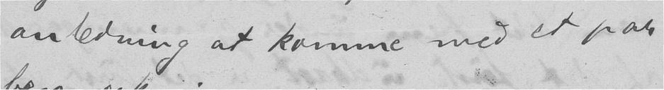anledning at komme med et par
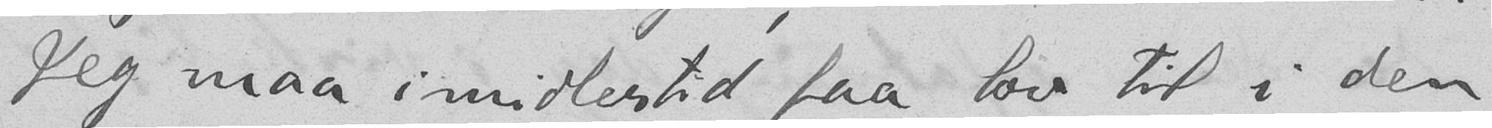Jeg maa imidlertid faa lov til i den
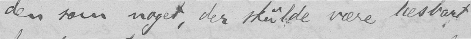den som noget, der skulde være læsbart
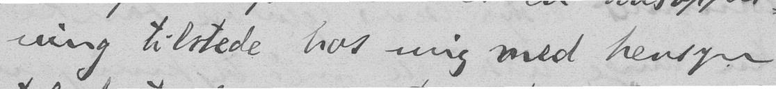ning tilstede hos mig med hensyn
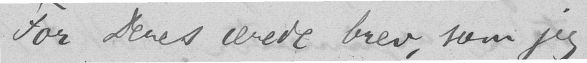For Deres ærede brev, som jeg
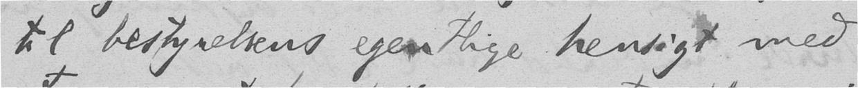til bestyrelsens egentlige hensigt med
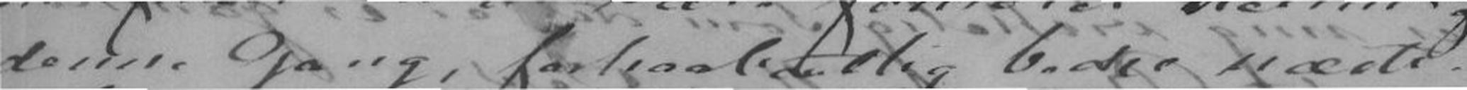denne Gang, forhaabentlig bedre næste.
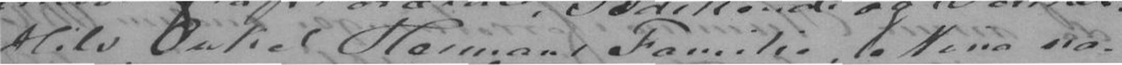Hils Onkel Hermans Familie, Nina na-
